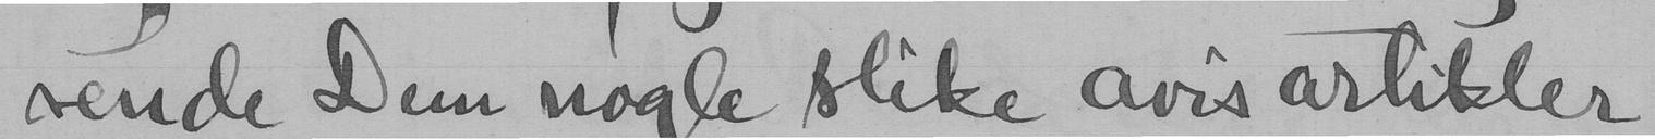sende Dem nogle slike avis artikler
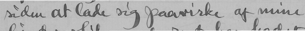siden at lade sig paavirke af mine
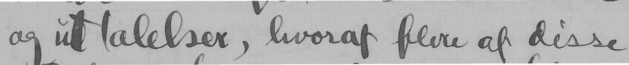og uttalelser, hvoraf flere af disse
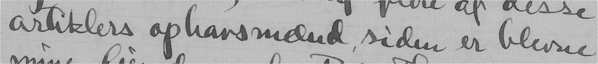artiklers ophavsmænd siden er blevne
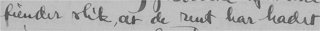fiender slik, at de rent har hadet
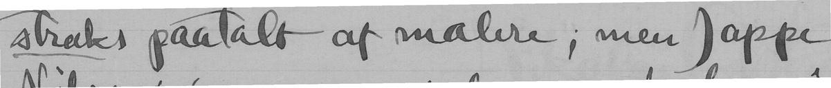straks paatalt af malere; men Jappe
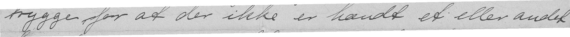trygge for at der ikke er hændt et eller andet
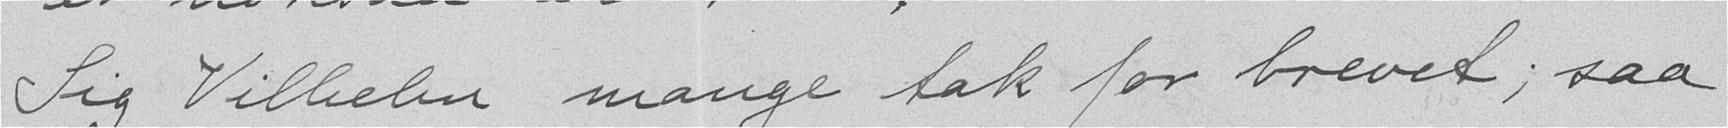Sig Vilhelm mange tak for brevet; saa
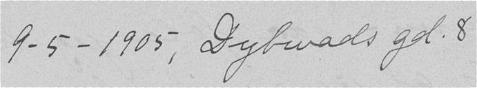9-5-1905, Dybwads gd. 8
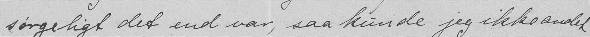sørgeligt det end var, saa kunde jeg ikke andet
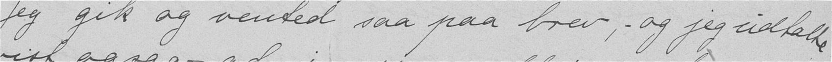Jeg gik og vented saa paa brev, - og jeg udtalte
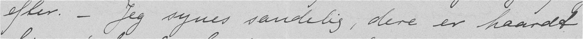efter. - Jeg synes sandelig, dere er haardt
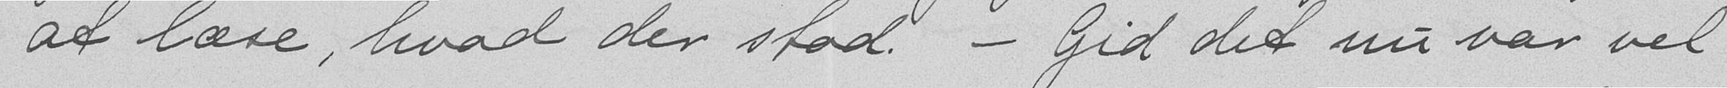at læse, hvad der stod. - Gid det nu var vel
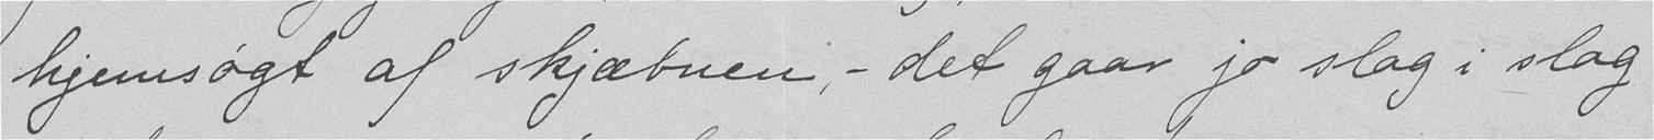hjemsøgt af skjæbnen, - det gaar jo slag i slag
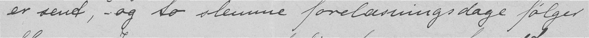er sent, - og to slemme forelæsningsdage følger
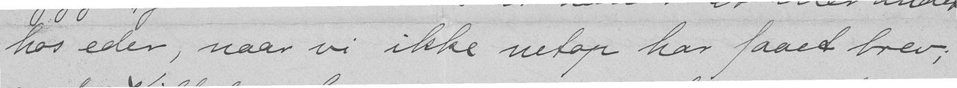hos eder, naar vi ikke netop har faaet brev;
